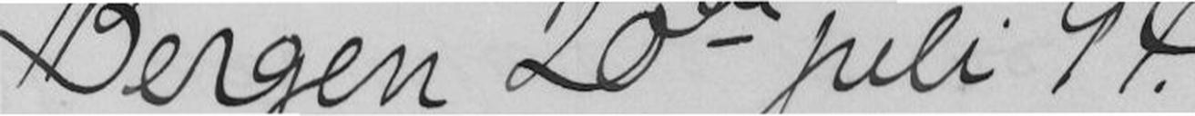Bergen 20 de juli 94.
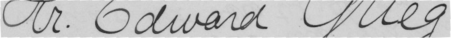Hr. Edward Grieg
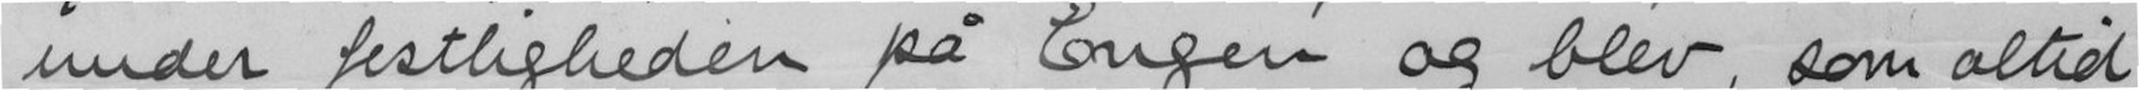under festligheden på Engen og blev, som altid
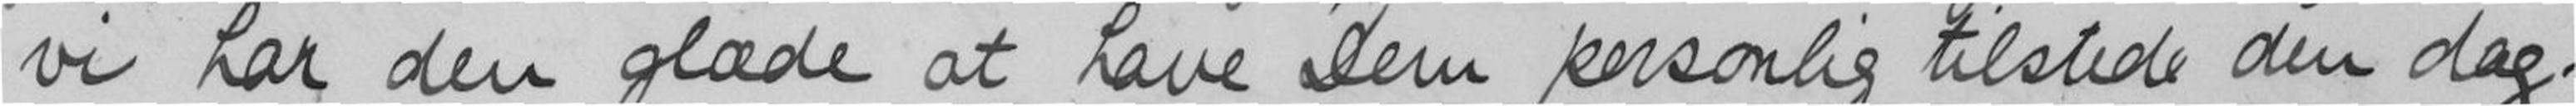vi har den glæde at have Dem personlig tilstede den dag.
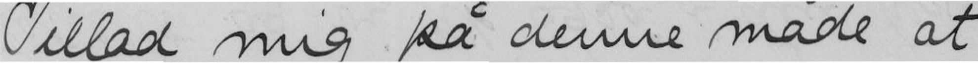Tillad mig på denne måde at
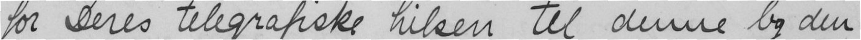for Deres telegrafiske hilsen til denne by den
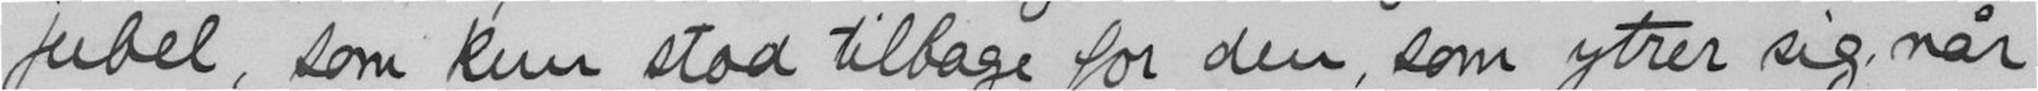jubel, som kun sto tilbage for den, som ytrer sig, når
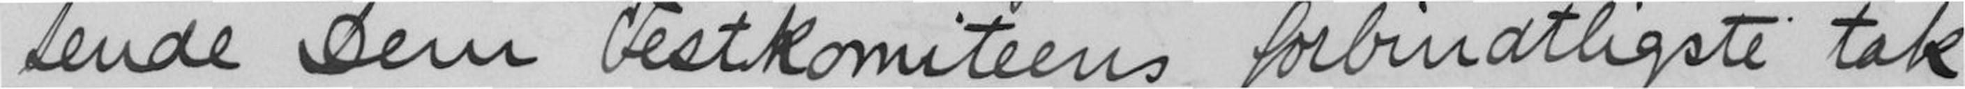sende Dem Festkomiteens forbindtligste tak
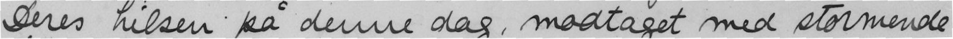Deres hilsen på denne dag, modtaget med stormende
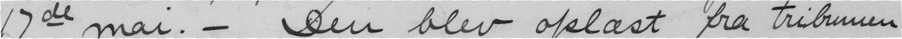17de mai.- Den blev oplæst fra tribunnen
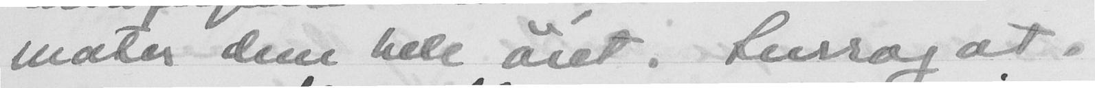mater dem hele året. R sajat.
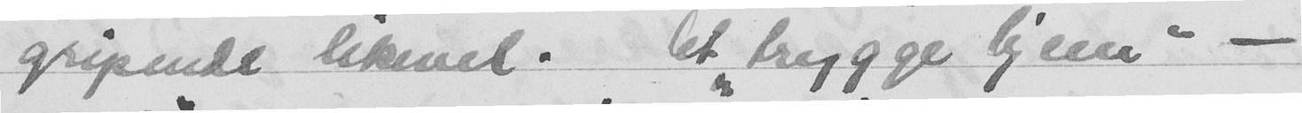gripende likevel. Det "trygge hjem" -
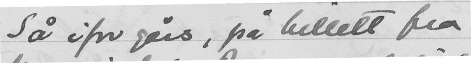Så ifor gårs, på billett fra
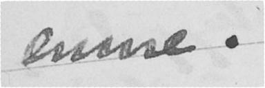emne.
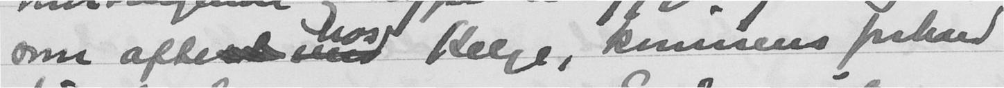som ofte hos Helge, kvinnenes forhold
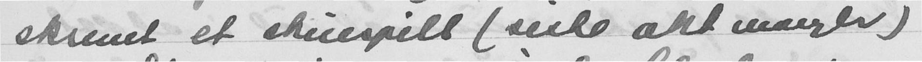skrevet et skuespill (siste akt mangler)
